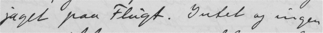jaget paa Flugt. Jutet og ingen
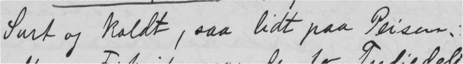Surt og kaldt, saa lidt paa Peisen
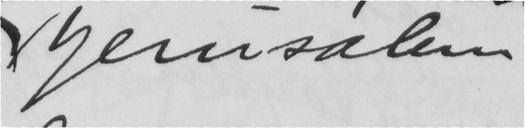Jerusalem
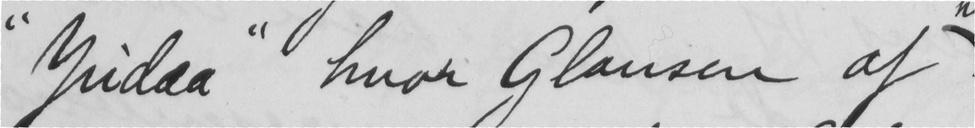"Judaa" hvor Glansen af
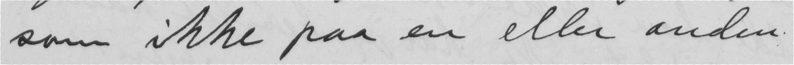som ikke paa en eller anden
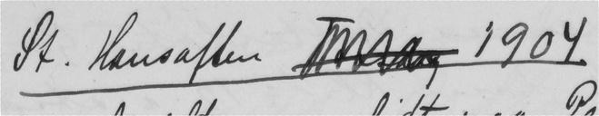St. Hansaften  1904
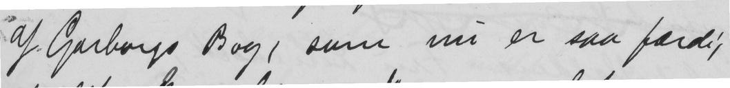af Garborgs Bog, som nu er saa færdig
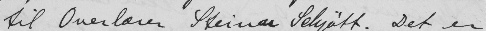til Overlærer Steinar Schjøtt. Det er
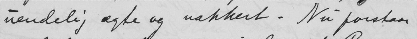uendelig ægte og vakkert. Nu forstaar
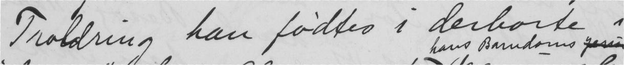Troldring han fødtes i derborte i
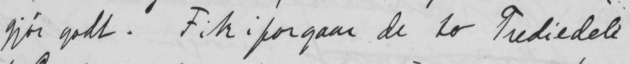gjør godt. Fik iforgaar de to Trediedele
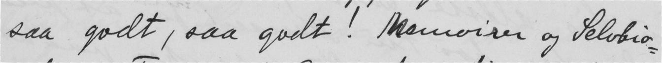saa godt, saa godt! Memoirer og Selvbio-
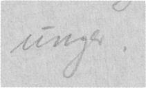unger .
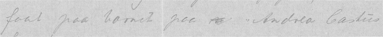faat paa barnet paa  "Andrea Castus
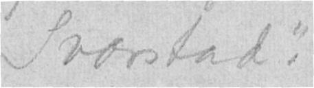Svarstad".
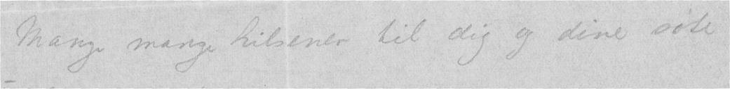Mange mange hilsener til dig og dine søte
